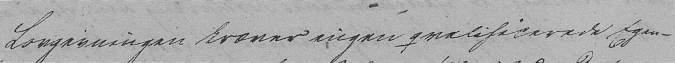Lovgivningen kræver ingen qvalificerede Egen-
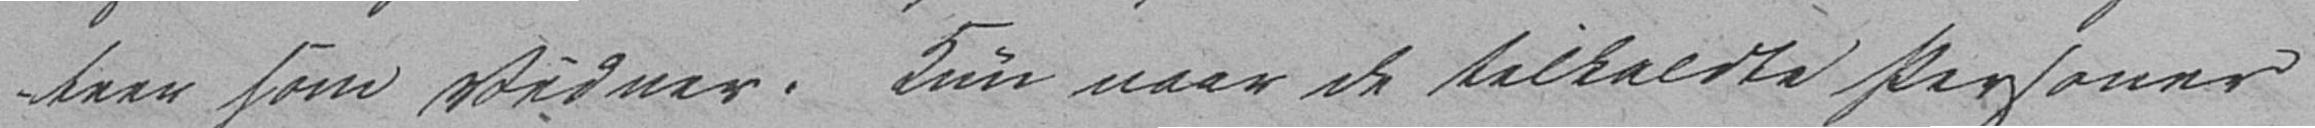teer som Vidner. Kun naar de tilkaldte Personer
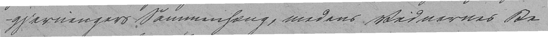gjerningers Sammenhæng, medens Vidnernes Be-
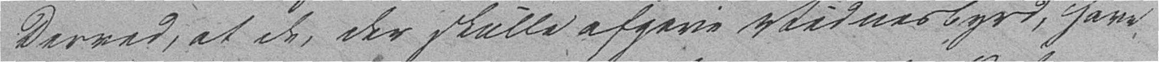Derved, at de, der skulle afgive Vidnesbyrd, have
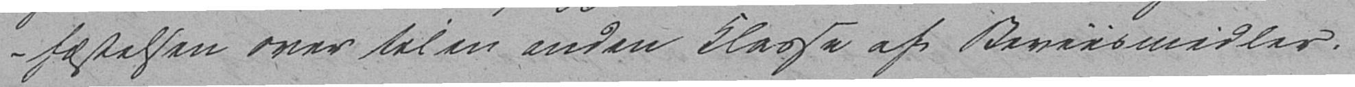fæstelsen over til en anden klasse af Beviismidler.
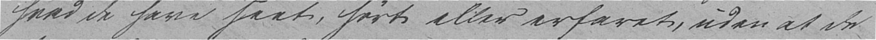hvad de have seet, hørt eller erfaret, uden at de
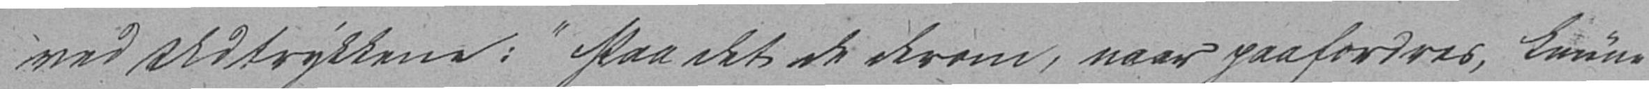ved Udtrykkene: "Paa det de derom, naar paafordres, kunne
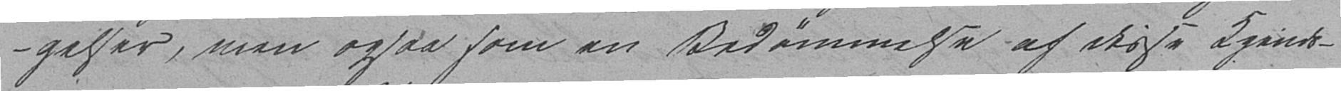gelser, men ogsaa som en Bedømmelse af disse Kjends-
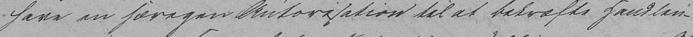have en særegen Autorisation til at bekræfte Handlin-
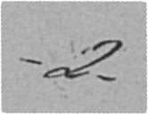- 2 -
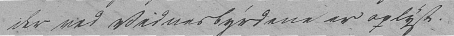der ved Vidnesbyrdene er oplyst.
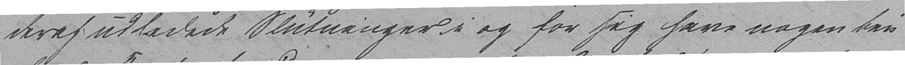deraf udledede Slutninger i og for sig have nogen bin-
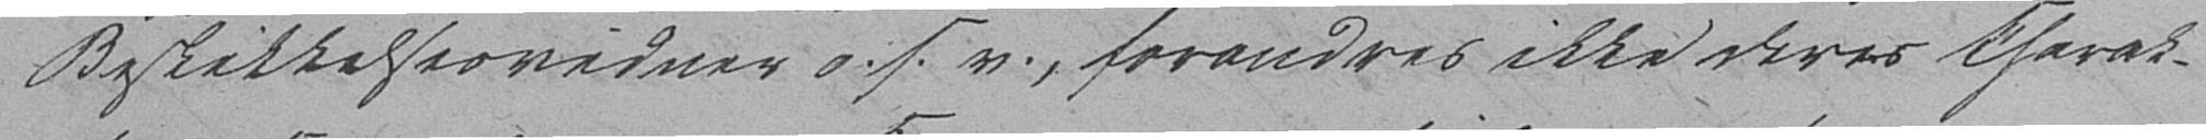Bestikkelsesvidner o. s. v., forandres ikke Deres Charak-
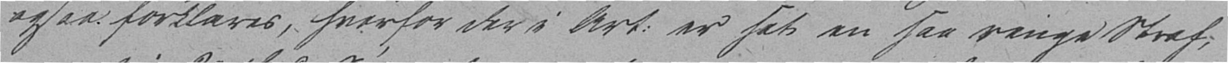ogsaa forklares, hvorfor der i Art. er sat en saa ringe Straf,
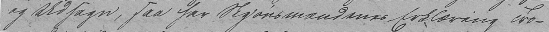og Udsagn, saa har Skjønsmændenes Erklæring Tro-
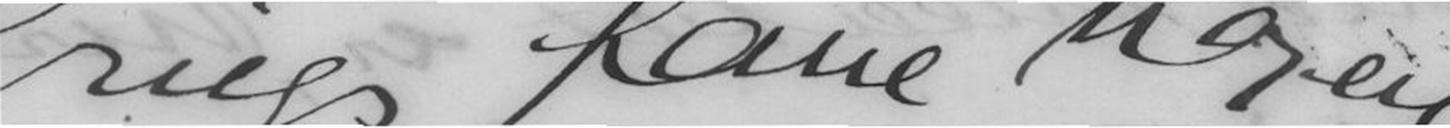Griegs fane højere
